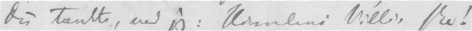Du tænkte, ved jeg: Himlens Villie ske!
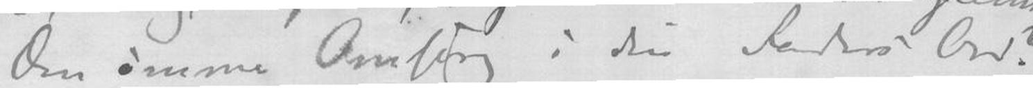Om ømme Omsorg i din Faders Ord?
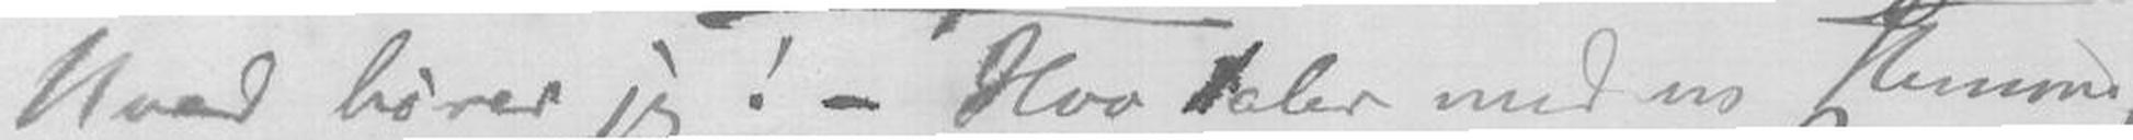Hvad hører jeg! - Hva taler med os Stemme,
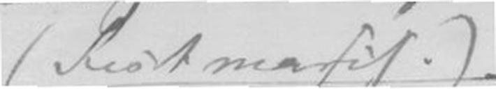Festmarsch.
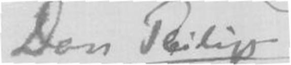Don Philip
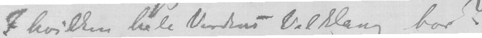I hvilken hele Verdens Vilklang bor?
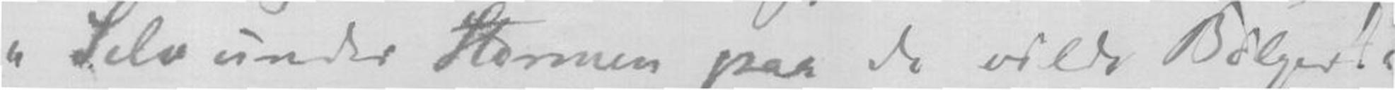"Selv under Stormen paa de vilde Bølger!
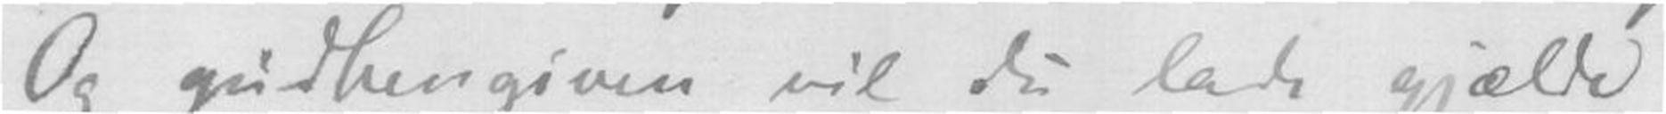Og gudhengiven vil du lade gjælde
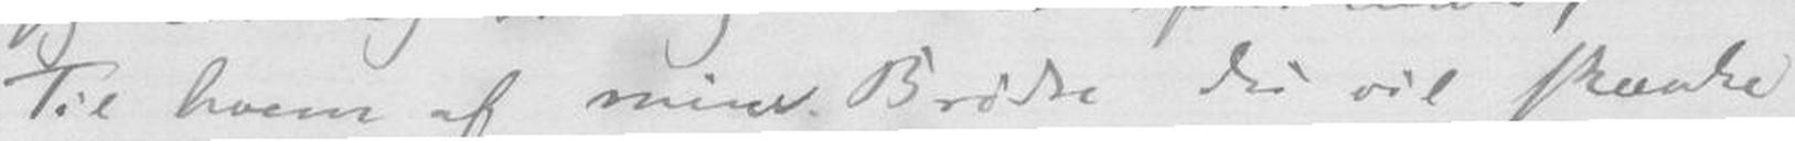Til hvem af mine Brødre du vil skænke
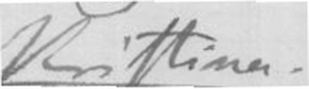Kristina.
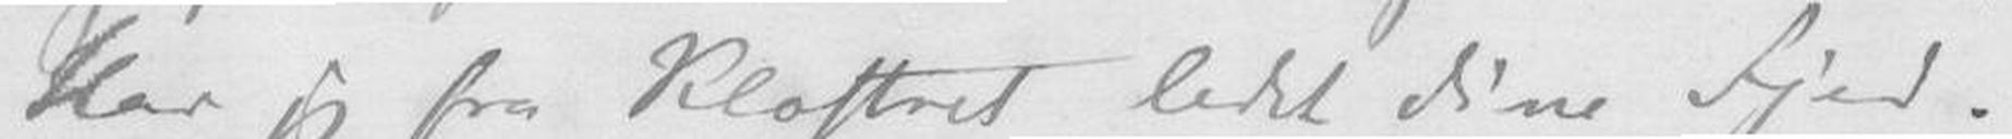Har jeg fra Klostret ledet dine Fjed.
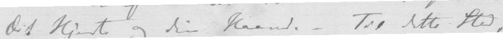Dit Hjerte og sin Haand. - Til dette Sted,
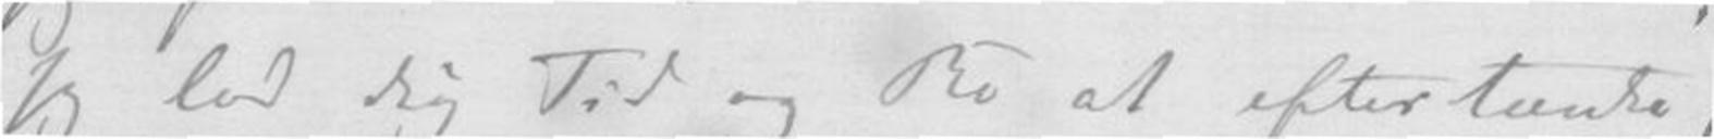Jeg lod dig Tid og Ro at eftertænke,
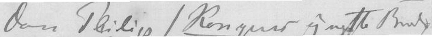Don Philip/Kongens yngste Brud
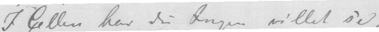I Cellen har du Ingen villet se,
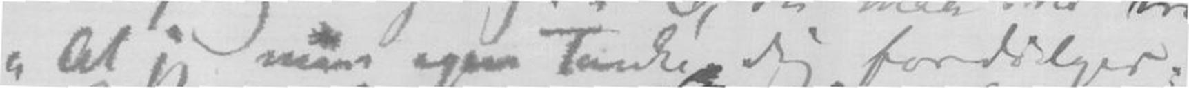"At jeg naar egen Tanke dig fordølger;
